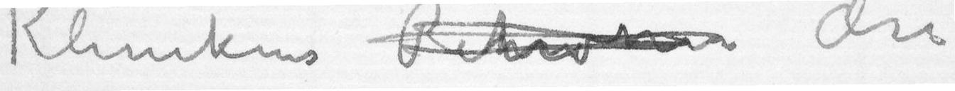Klinikens R Ære
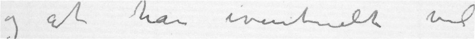og at han eventuelt vil
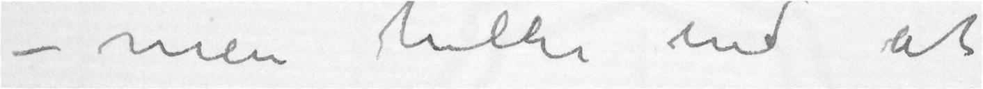– Men heller end at
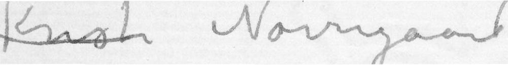Kristi Nørregaard
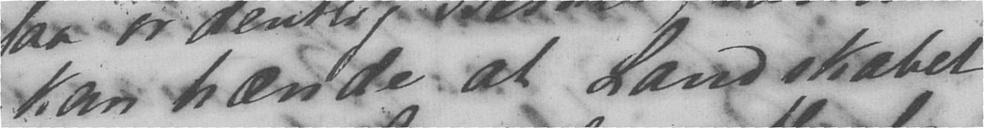kan hænde at Landskabet
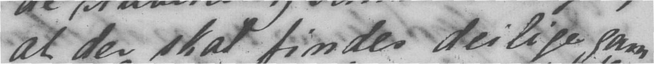at der skal findes deilige gam-
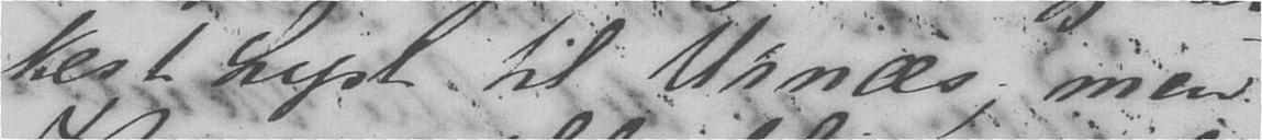kest Lyst til Urnæs; men
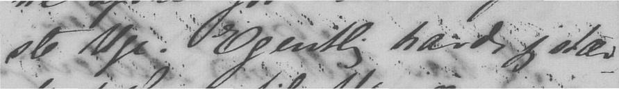ste Uge. Egentlig havde jeg stær-
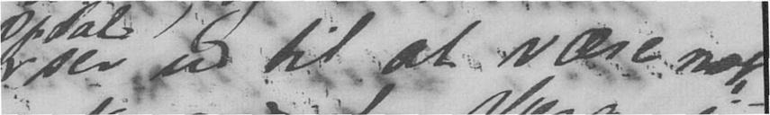ser ud til at være nok-
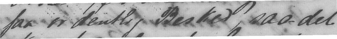faa ordentlig Besked, saa det
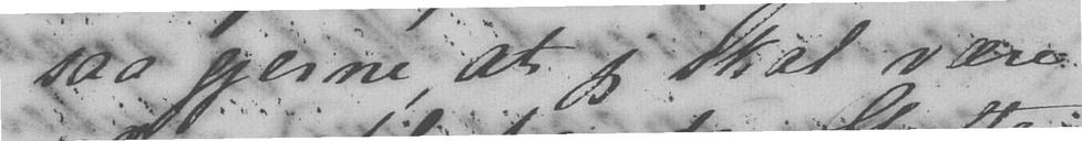saa gjerne at jeg skal være
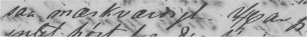saa mærkværdigt. Har jeg
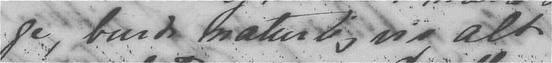ge, burde naturligvis alt
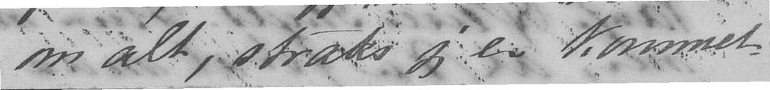om alt, straks jeg er kommet
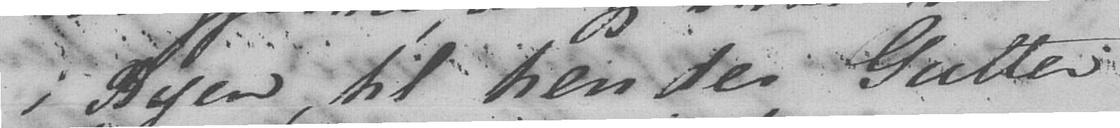i Byen, til hendes Gutter
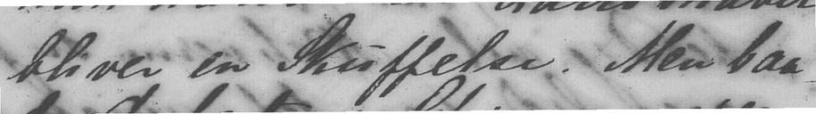bliver en Skuffelse. Men baa-
